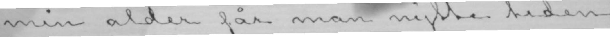min alder får man nytte tiden
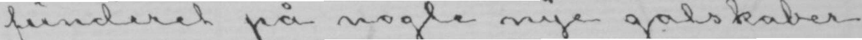funderet på nogle nye galskaber
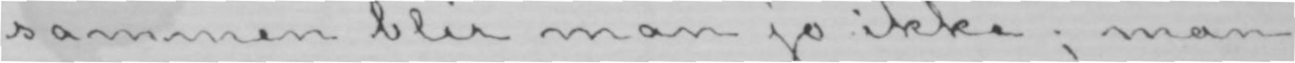sammen blir man jo ikke; man
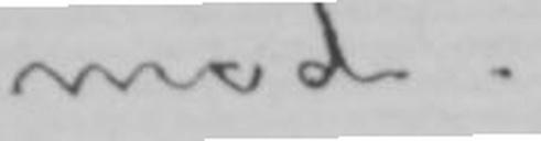mod.-
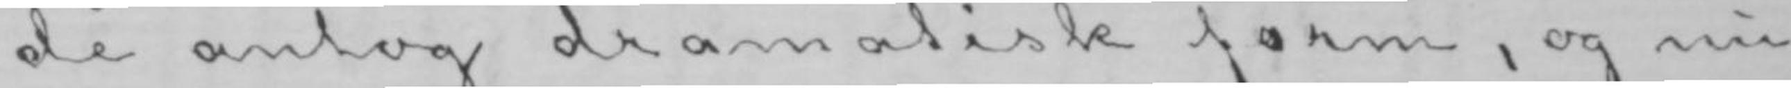de antog dramatisk form, og nu
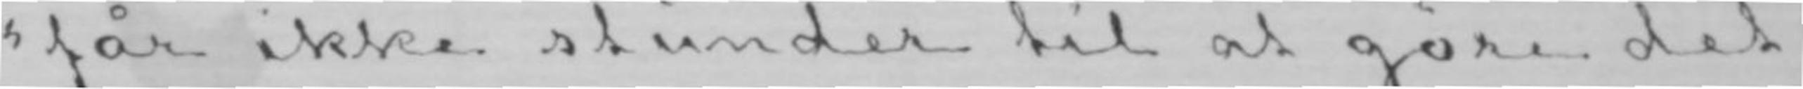«får ikke stunder til at gøre det
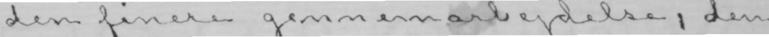den finere gennemarbejdelse, den
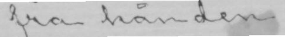fra hånden.
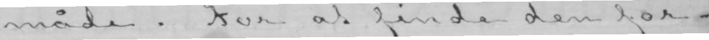måde. For at finde den for-
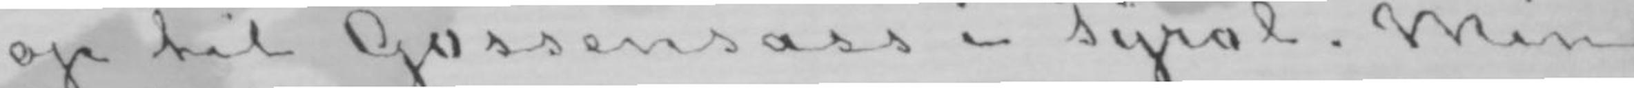op til Gossensass i Tyrol. Min
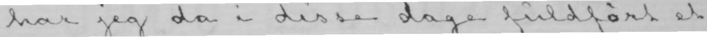har jeg da i disse dage fuldført et
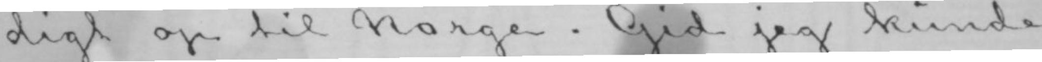digt op til Norge. Gid jeg kunde
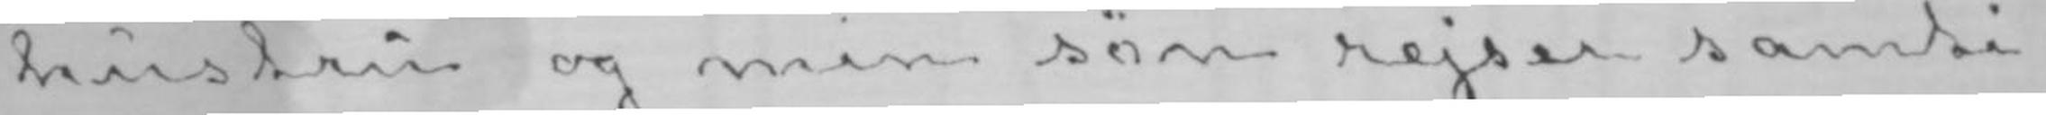hustru og min søn rejser samti-
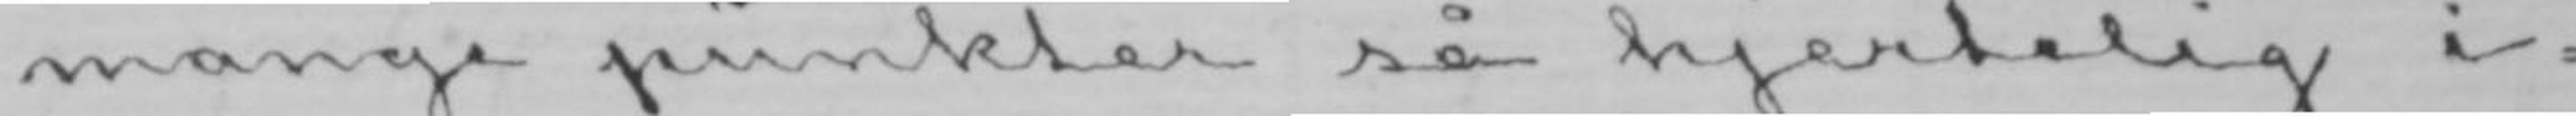mange punkter så hjertelig i-
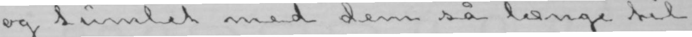og tumlet med dem så længe til
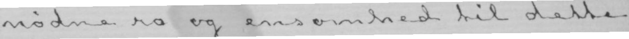nødne ro og ensomhed til dette
